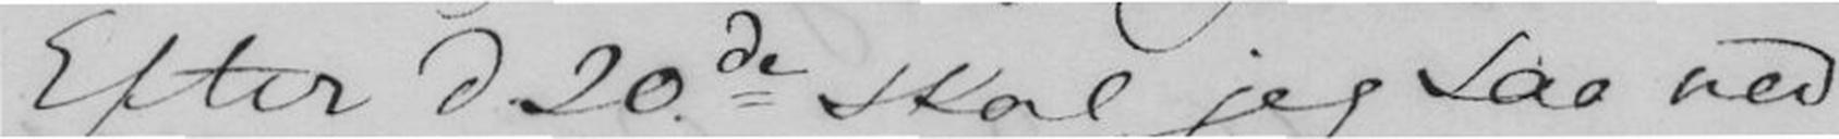Efter d 20de skal jeg saa ned
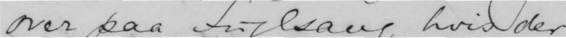over paa Fuglsang, hvis der
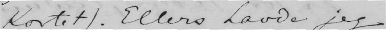Kortet). Ellers havde jeg
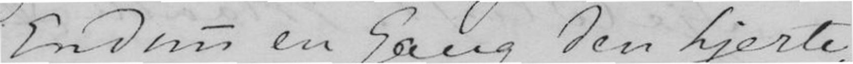Endnu en Gang den hjerte-
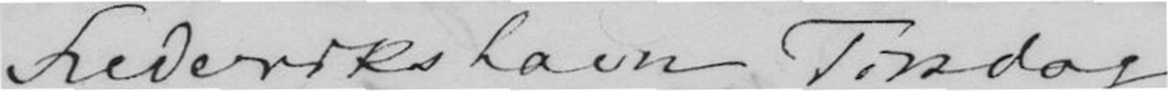Frederikshavn Tirsdag
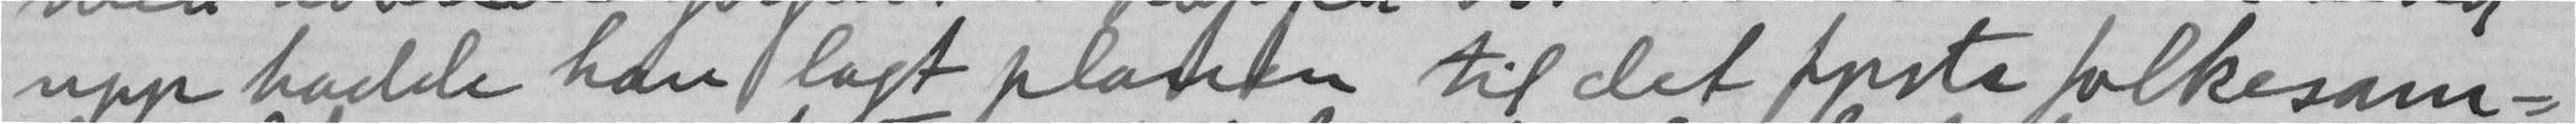upp hadde han lagt planen til det fyrste folkesam-
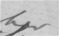har
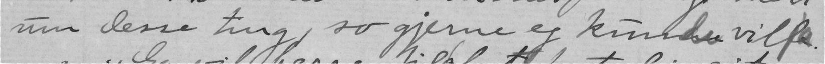um desse ting, so gjerne eg kunde ville.
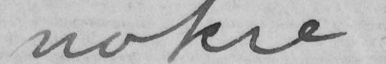nokre
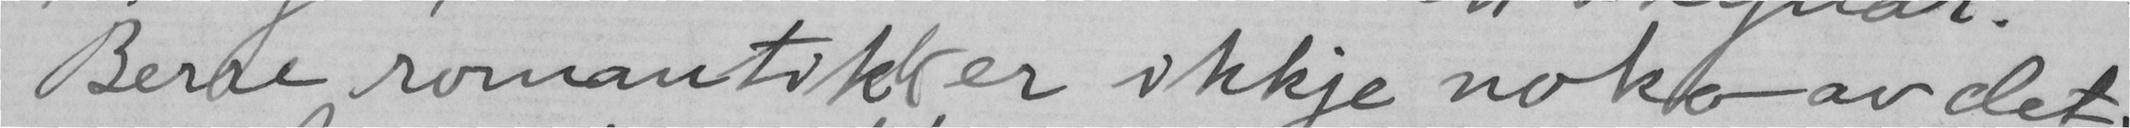Berre romantik er ikkje noko av det,
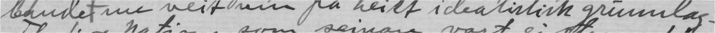bandet me veit um på heilt idealistisk grunnlag -
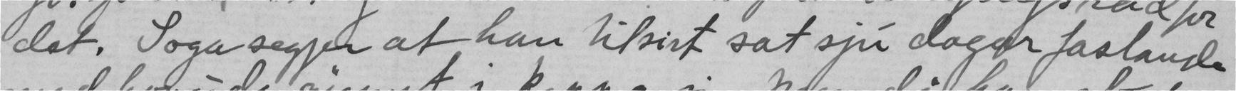det. Soga segjer at han tilsist sat sju dagar fastande
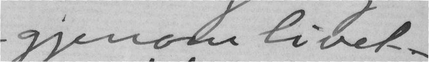gjenom livet -
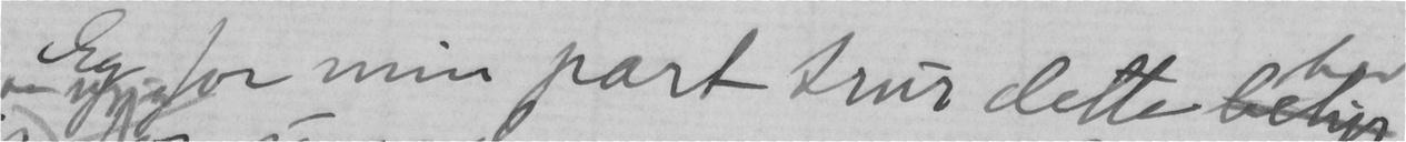Eg for min part trur dette betyr
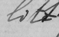litt
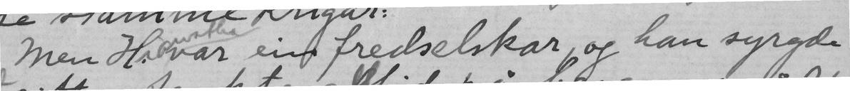Men H. var ein fredselskar, og han syrgde
